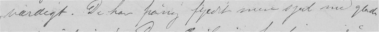værdigt. De har påny fyldt min sjæl med glæde
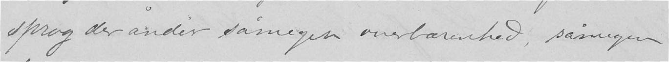sprog der ånder såmegen overbærenhed, såmegen
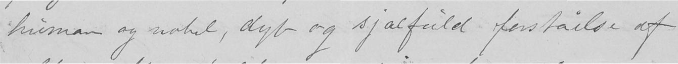human og nobel, dyb og sjælfuld forståelse af
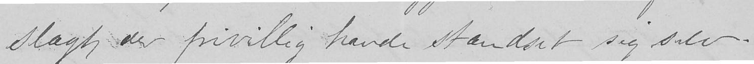slægt der frivillig havde standset sig selv.
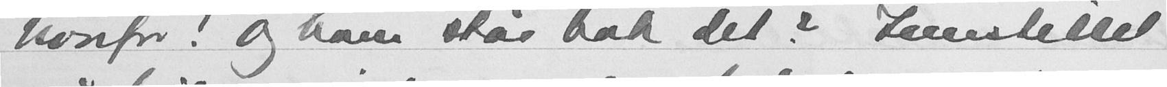hvorfor! Og han står bak det? Innstillet
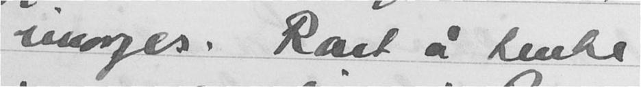imorges. Rart å tenke
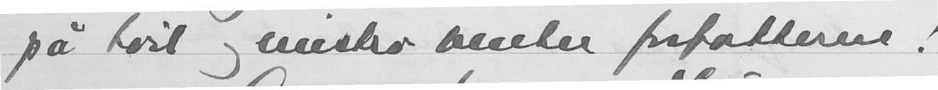på tvil og mistro venter forfatterne!
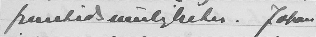og fremtidsmuligheter. Johan
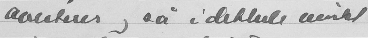avestees og så i dethele mørkt
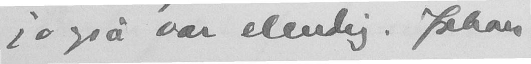jo også var elendig. Johan
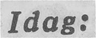Idag:
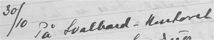30/10 På Svalbard-kontoret
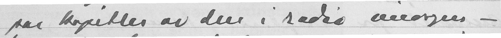par kapitler av den i radio imorgen -
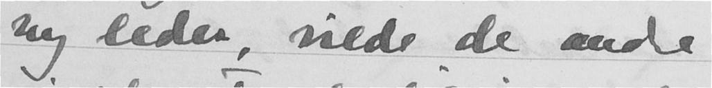ny leder, vilde de andre
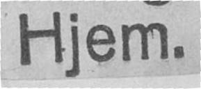Hjem.
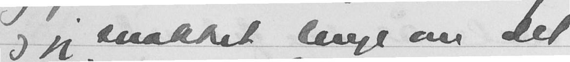og jeg snakket lenge om det
